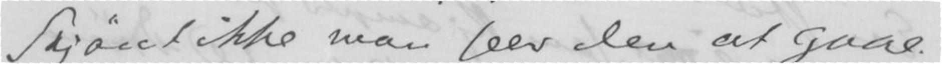Skjønt ikke man seer den at gaae.
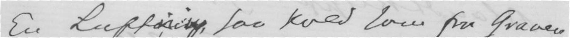En Lufføring, saa kved som fra Graven
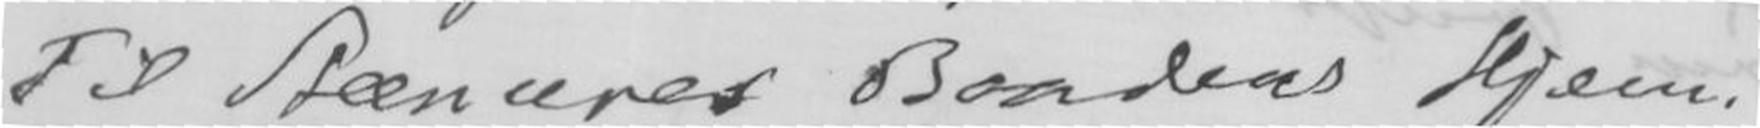Til Steenurer Baadens Hjem.
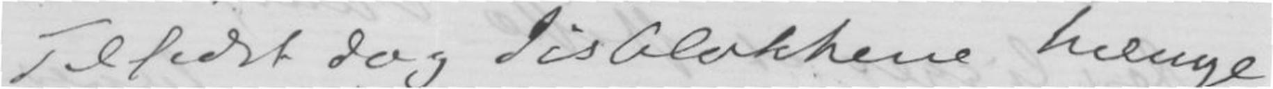Tilsidst dog Iisblokkene hænge
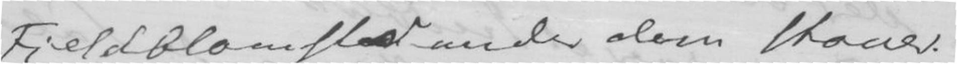Fjeldblomster under dem staaer.
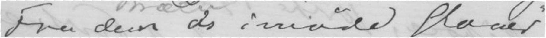Fra dem de imøde slaaer
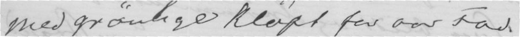Med grønlige kløft for over Fod.
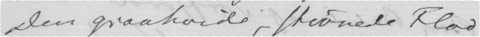Den graahvide, stivnede Flod -
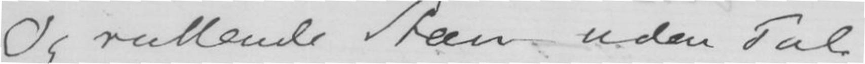Og rullende Steen uden Tale.
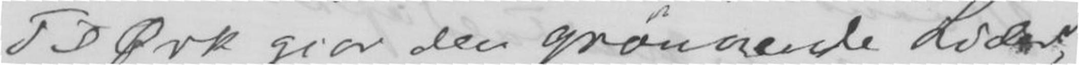Til Ørk gier den grønnende Lider,
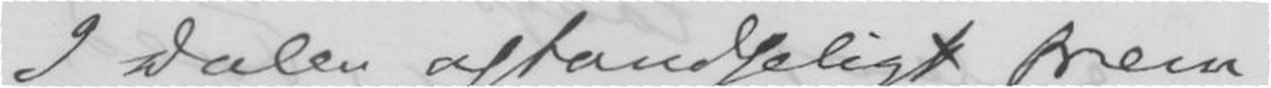I dalen ustandseligt frem,
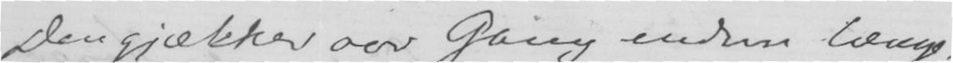Den gjækker over Gaing endnu længe
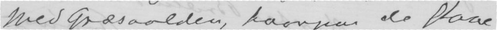Med Græsvolden, hvorpaa de staae
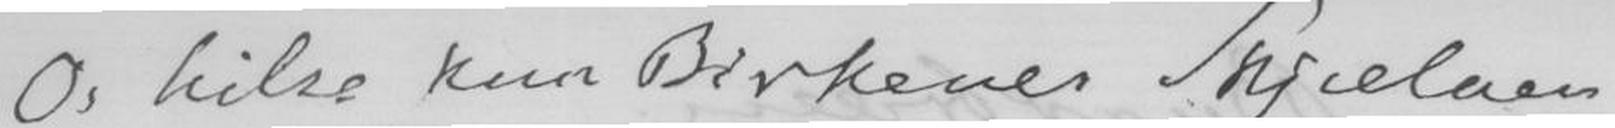Os hilse kun Birkenes Skjælven
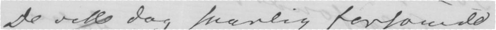De ville dog snarlig forsonede
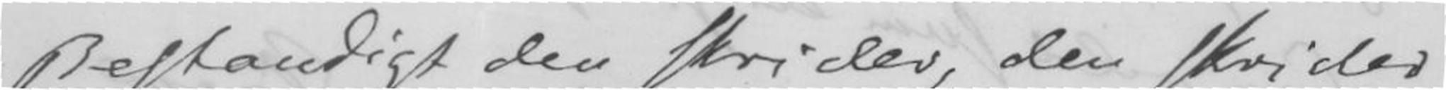Bestandigt den skrider, den skrider
